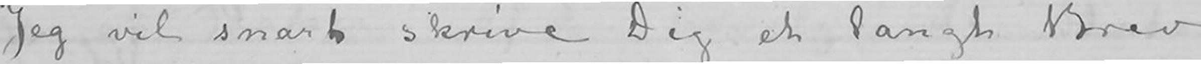Jeg vil snart skrive Dig et langt Brev
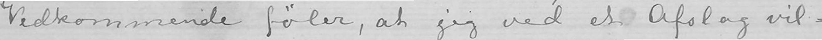Vedkommende føler, at jeg ved et Afslag vil-
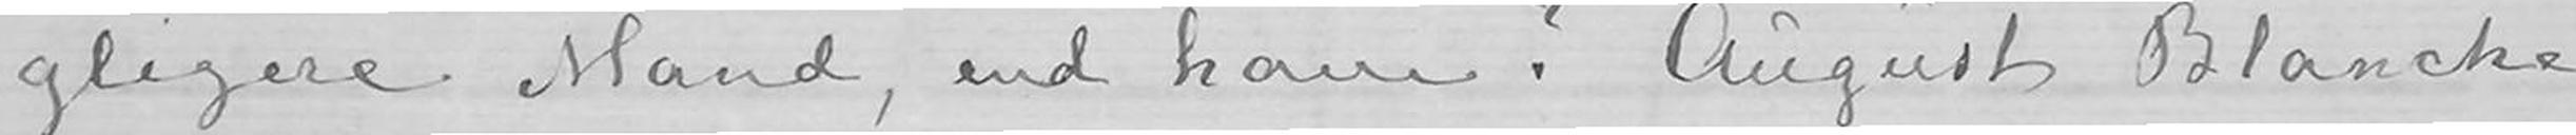gligere Mand, end ham? August Blanche
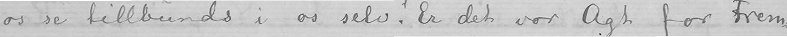os se tillbunds i os selv! Er det vor Agt for Frem-
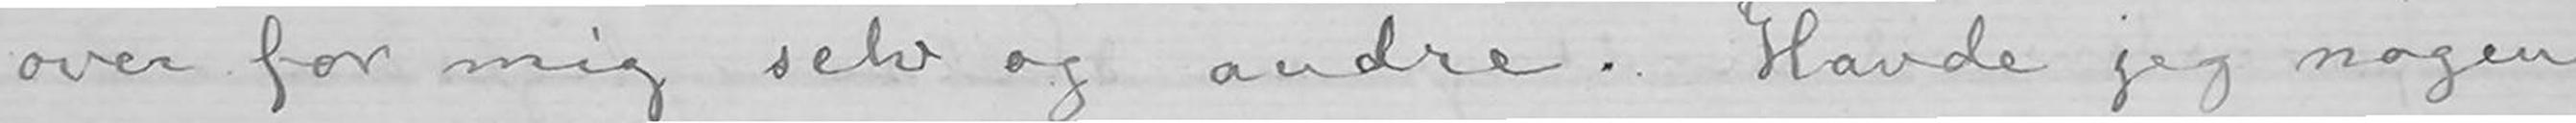over for mig selv og andre. Havde jeg nogen
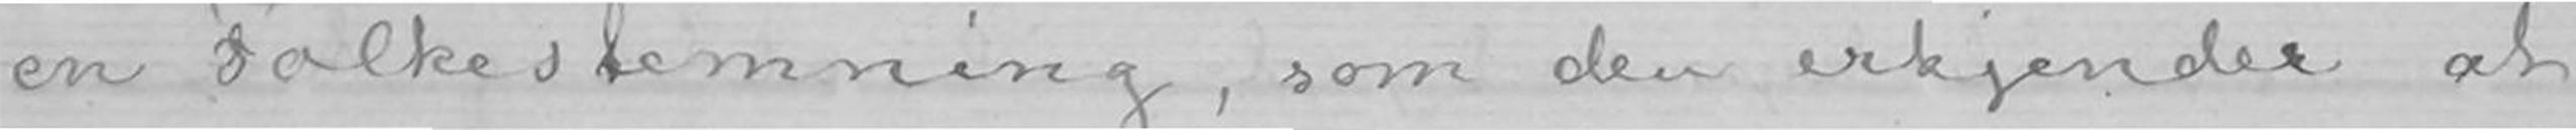en Folkestemning, som den erkjender at
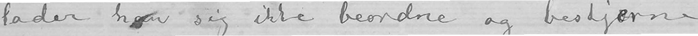lader han sig ikke beordne og bestjernebestj
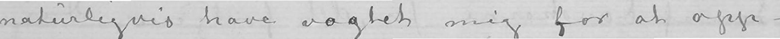naturligvis have vogtet mig for at opp-
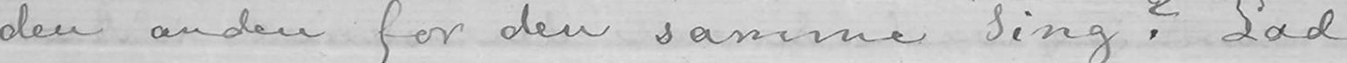den anden for den samme Ting? Lad
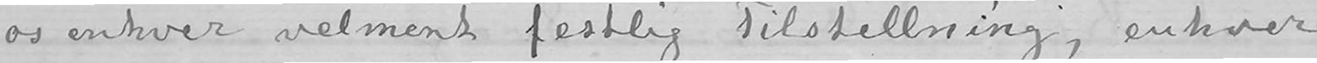os enhver velment festlig Tilstellning, enhver
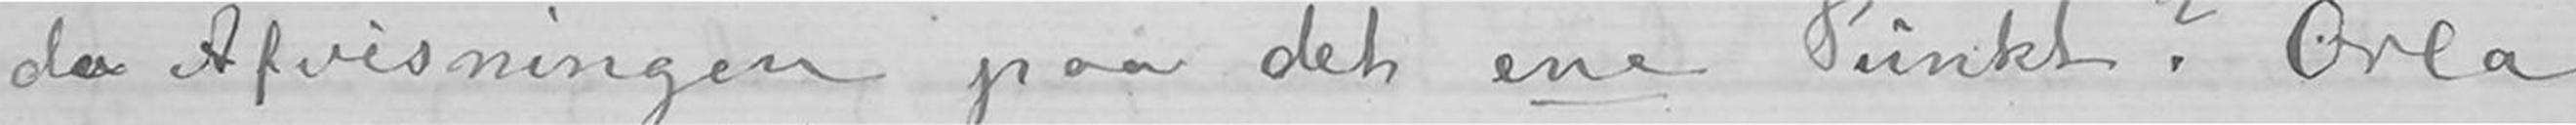da Afvisningen paa det ene Punkt? Orla
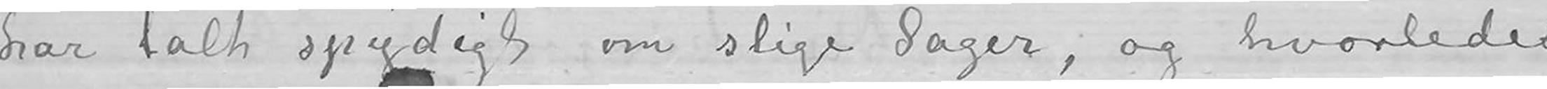har talt spydigt om slige Sager, og hvorledes
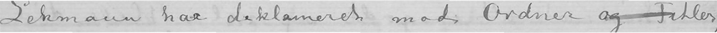Lehmann har deklameret mod Ordner og Titler;
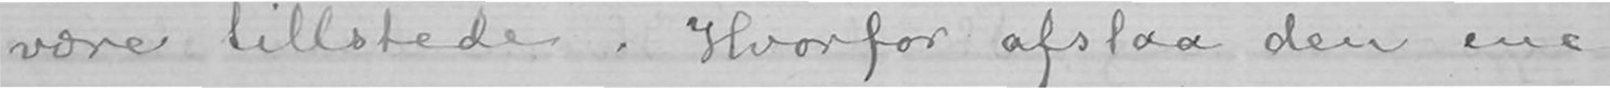være tillstede. Hvorfor afslaa den ene
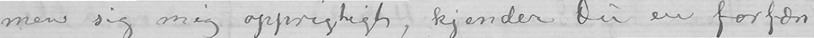men sig mig opprigtigt, kjender Du en forfæn-
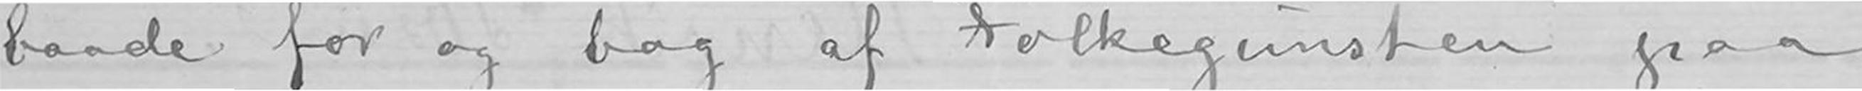baade for og bag af Folkegunsten paa
